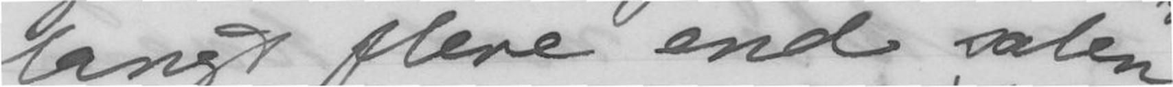langt flere end salen
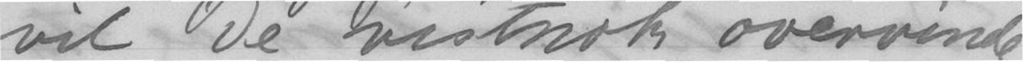vil De vistnok overvinde
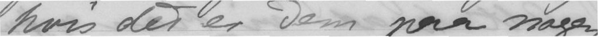hvis det er Dem paa nogen
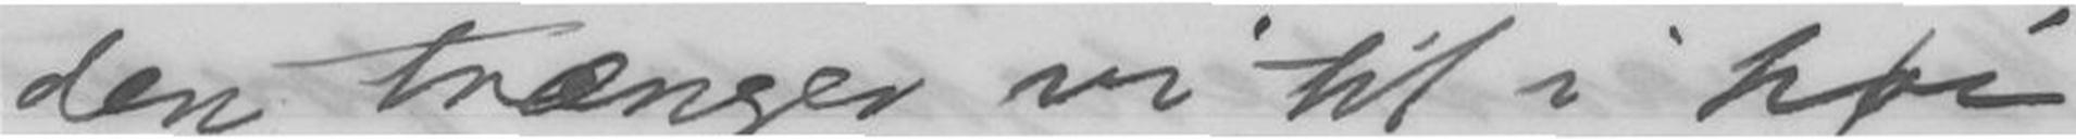den trænger vi til i høi
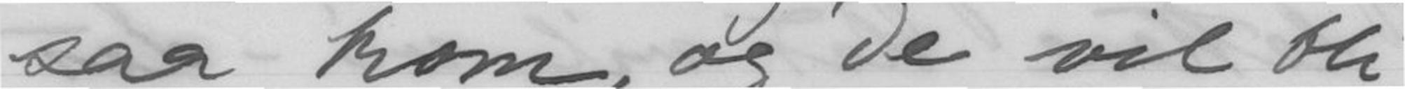saa kom, og De vil bli
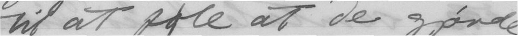til at føle at De gjorde
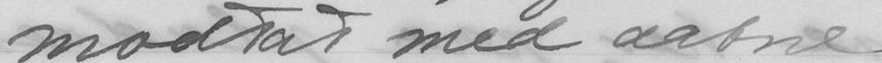modtat med aabne
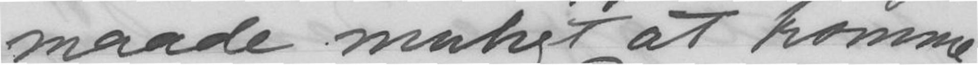maade muligt at komme
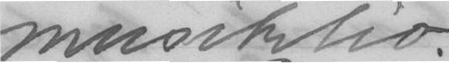musikliv.
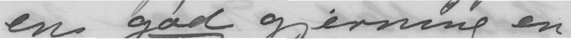en god gjerning en
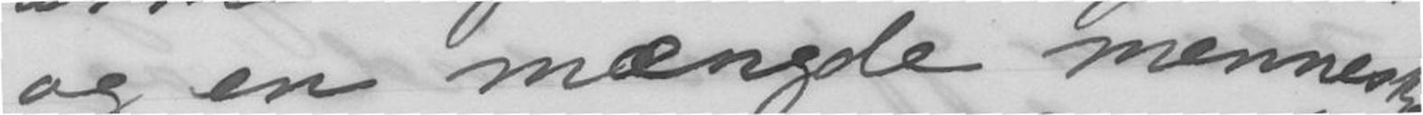og en mængde mennesker
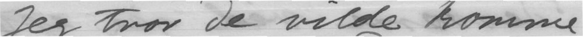Jeg tror De vilde komme
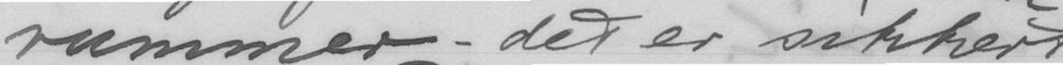rummer - det er sikkert.
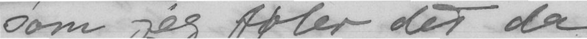som jeg føler det da
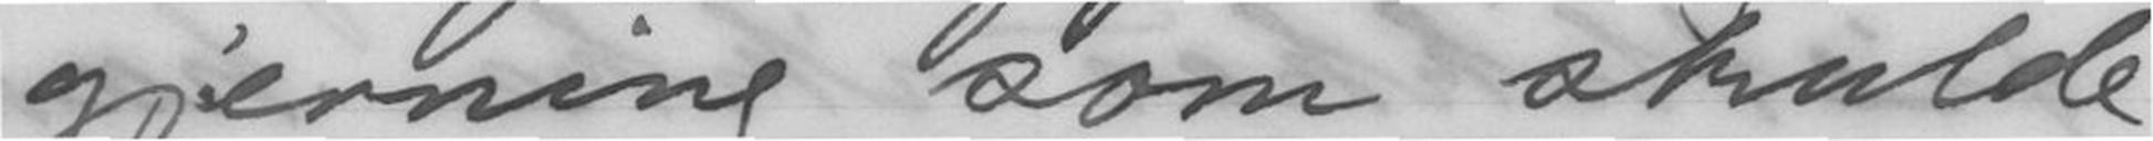gjerning som skulde
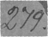279.
